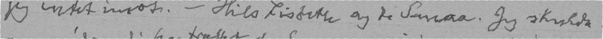jeg intet imot. - Hils Lisbeth og de Smaa. Jeg skulde
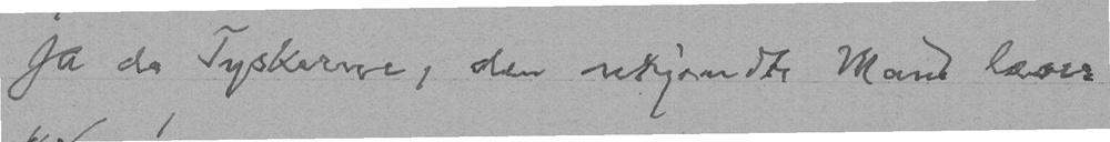Ja de Tyskerne, den ukjendte Mand læser
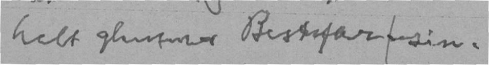helt glemmer Bestefar | sin.
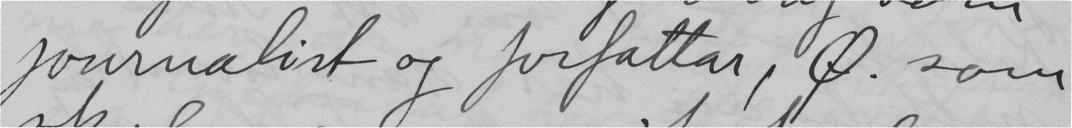journalist og forfattar, Ø. som
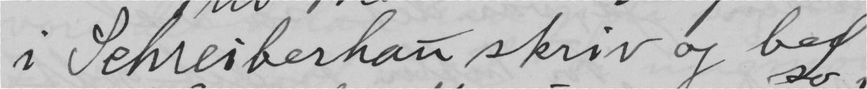i Schreiberhau skriv og beg
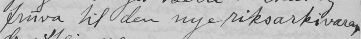fruva til den nye riksarkivaren
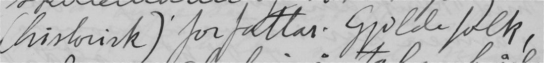(historisk) forfattar. Gjilde folk,
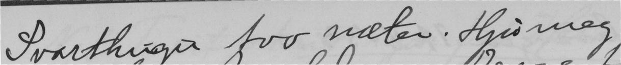Svarthugu tvo næter. Hjå meg
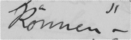können" –
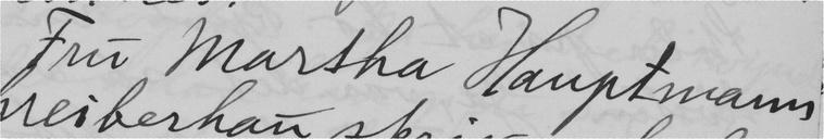Fru Martha Hauptmann
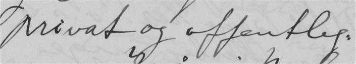privat og offentleg.
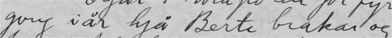gong i år hjå Berte brakar og
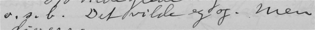o.s.b. Det vilde eg og. Men
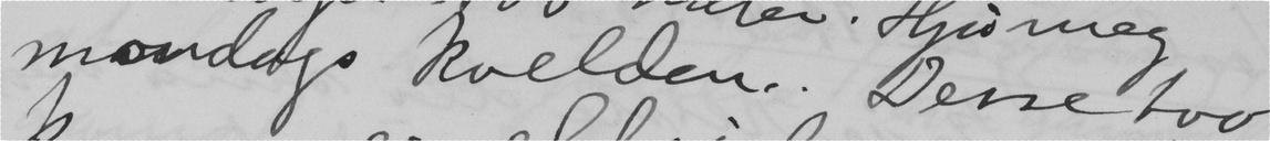mondags kvelden. Desse tvo
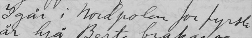Igår i Nordpolen for fyrste
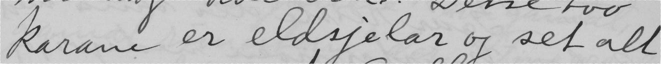karane er eldsjelar og set alt
